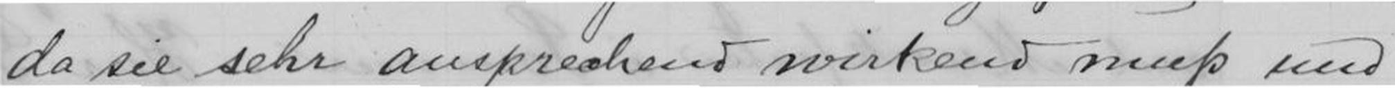da sie sehr ausprechend wirkend muss und
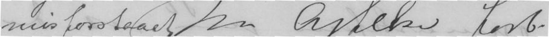misforstaaet. M Agtelse forb.
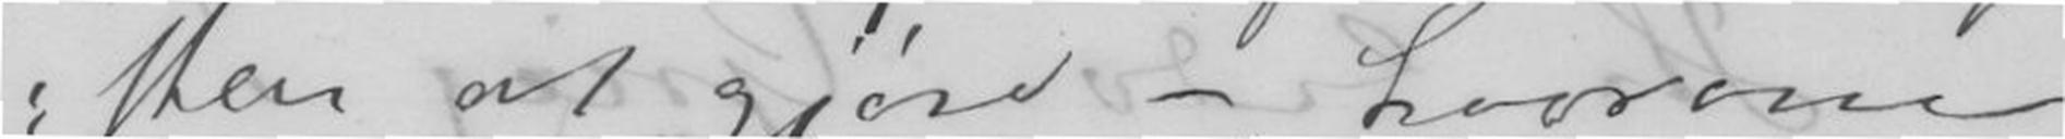sten at gjøre - hvorom
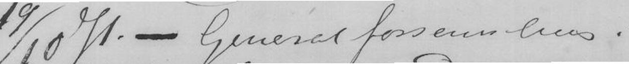19/10 71. - Generalforsamling i
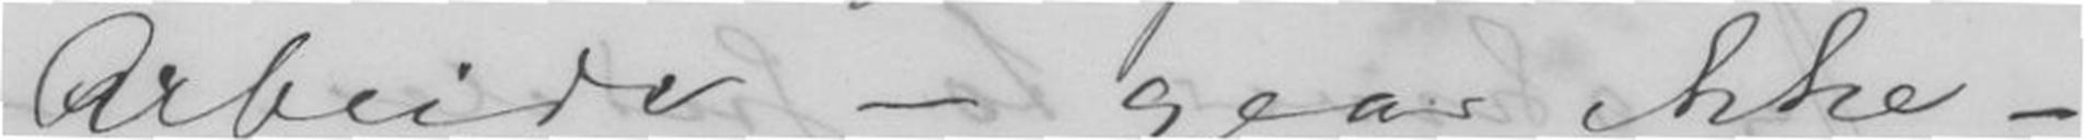Arbeide - gaar ikke -
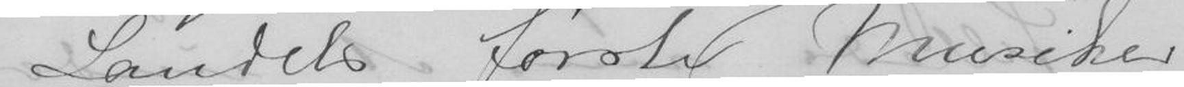Landets første Musiker
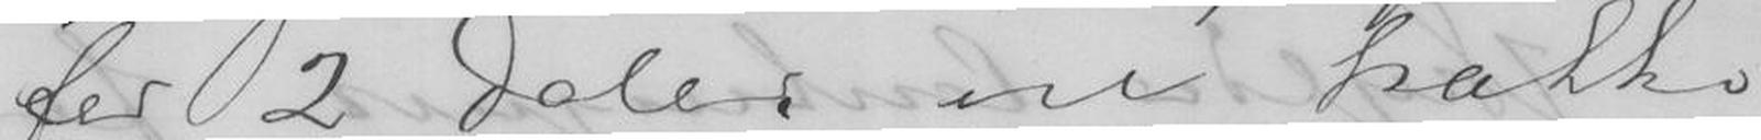for 2 Daler vil trække
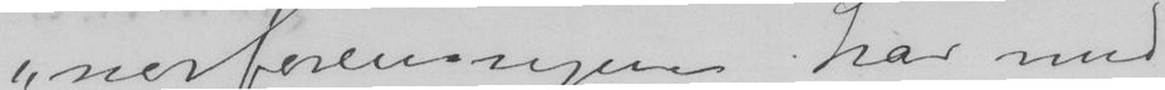=nerforeningen har med
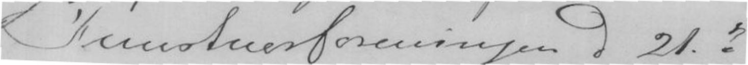Kunstnerforeningen d 21.de
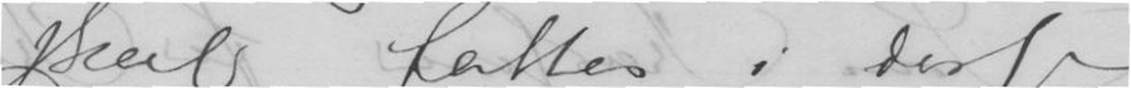skal fattes i disse
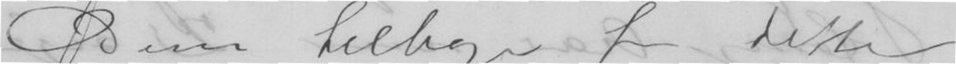Dem tilbage fra dette
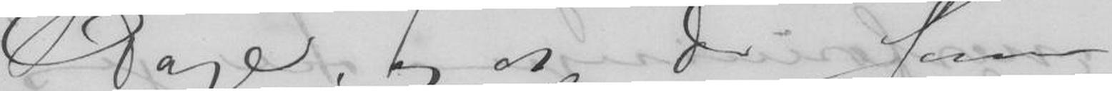Dage, og at de som
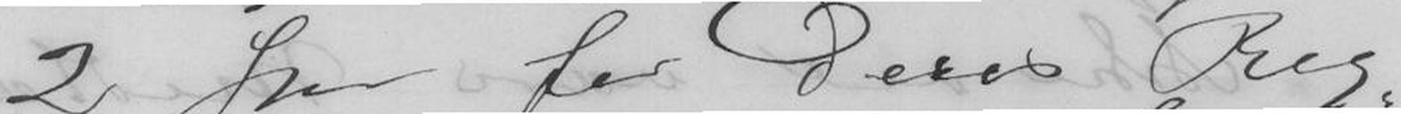2 Spd for Deres Reg-
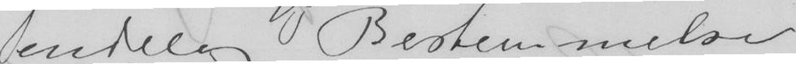endelig Bestemmelse
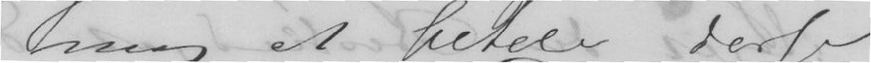mig at betale disse
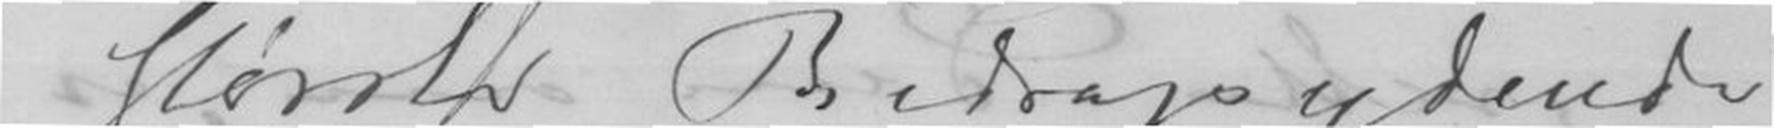og største Bidragsydende
